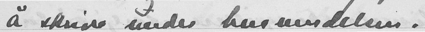å skrive under henvendelsen.
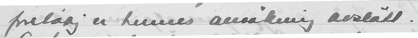foreløpig er hennes ansøkning avslått.
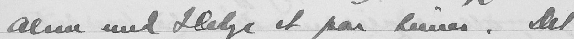alene med Helge et par timer. Det
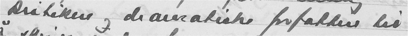kritikere og dramatiske forfattere til
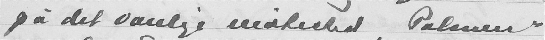på det vanlige møtested Palmen
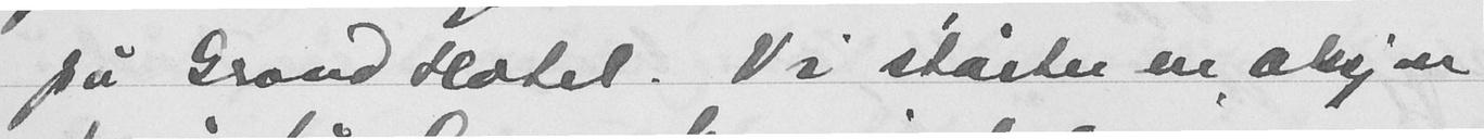på Grand Hotel. Vi starter en aksjon
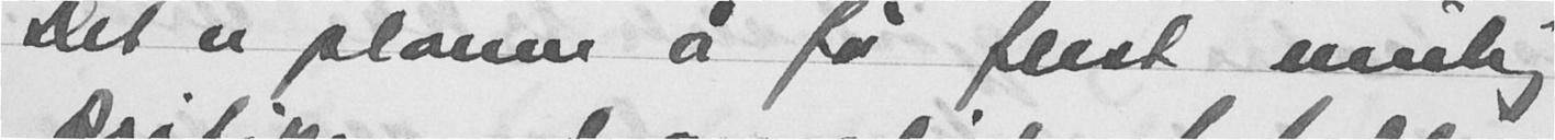Det er planen å få flest mulig
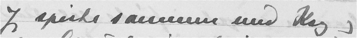Jeg spiste sammen med Krog og
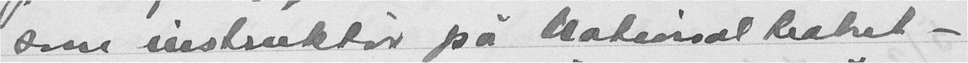som instruktør pa Nationalteatret -
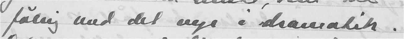føling med det nye i dramatik.
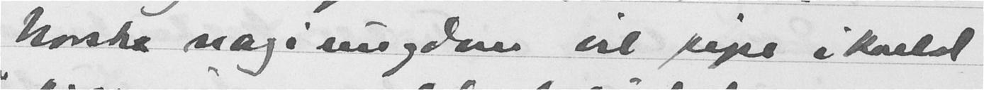Norske naziungdom vil pipe i kveld
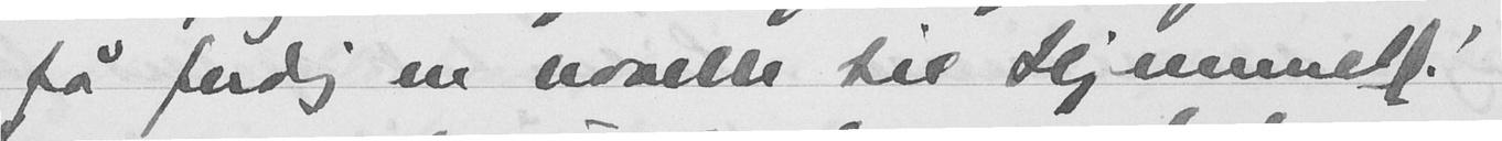få ferdig en novelle til Hjemmet(!
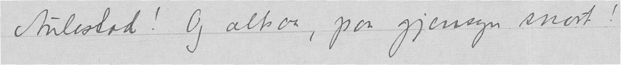Aulestad! Og altsaa, paa gjensyn snart!
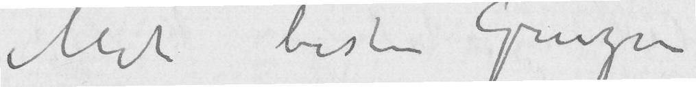Mit besten Gruzen
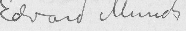Edvard Munch
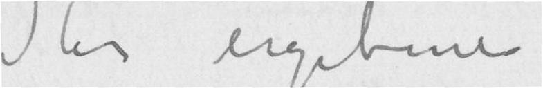Ihr ergebener
